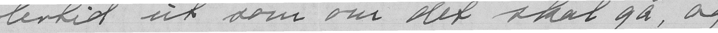lertid ut som om det skal gå, og
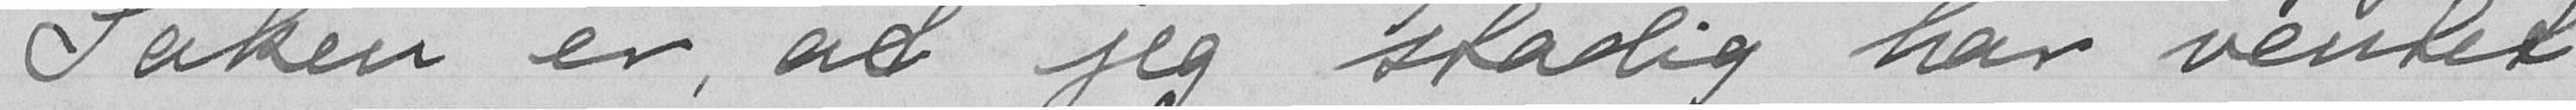Saken er, at jeg stadig har ventet
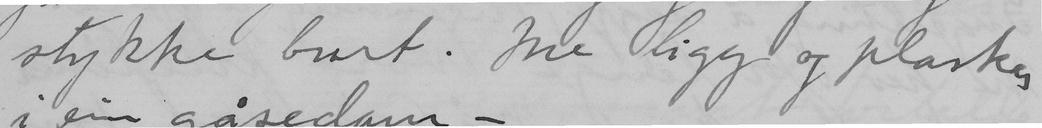stykke burt. Me ligg og plaske
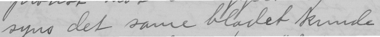syns det same bladet kunde
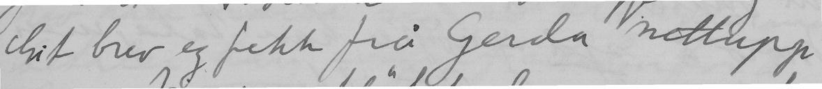Eit brev eg fikk frå Gerda nettupp
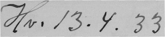Hv. 13.4.33
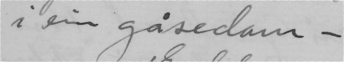i ein gåsedam –
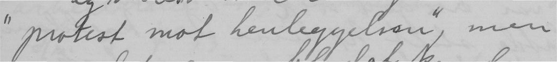"protest mot henleggelsen", men
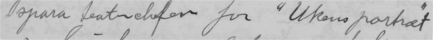spara teaterchefen for "Ukens portræt"
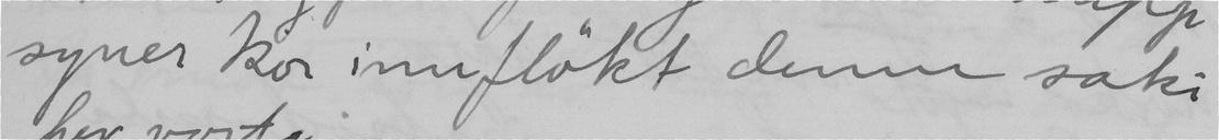syner kor innflöøkt denne saki
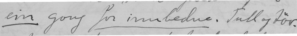ein gong for innbedne. Tull og töv.
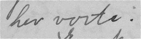hev vorte.
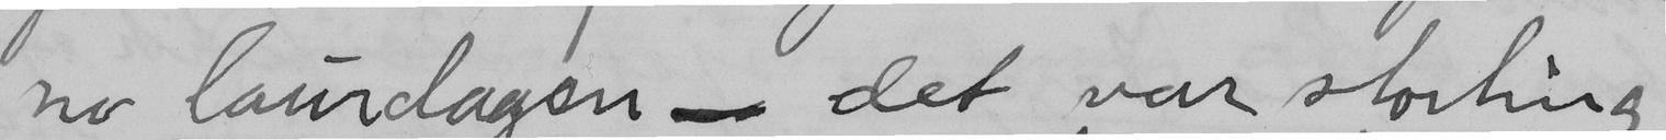no laurdagen – det var storting
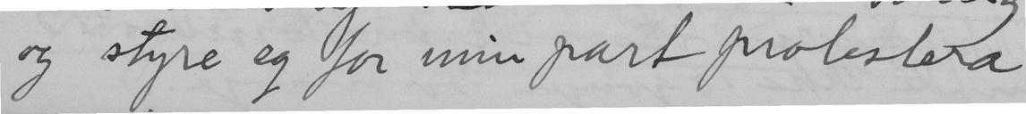og styre eg for min part protestera
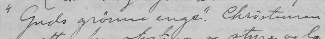"Guds grönne enge". Christensen
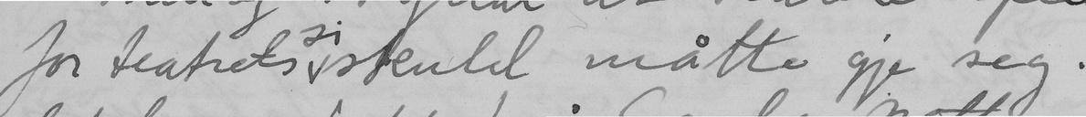for teatret si skuld måtte gje seg.
